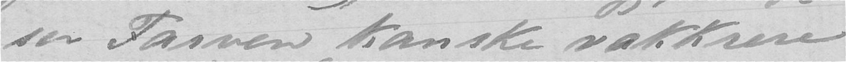ser Farven kanske vakkrere
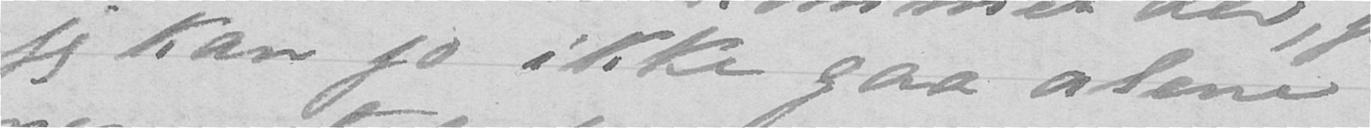jeg kan jo ikke gaa alene
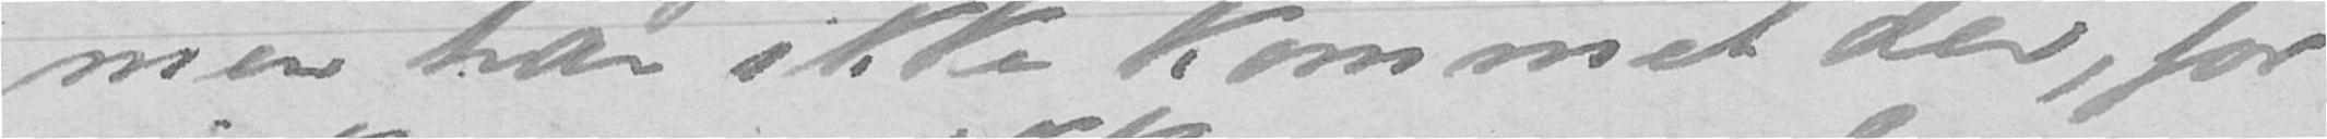men har ikke kommet der, for
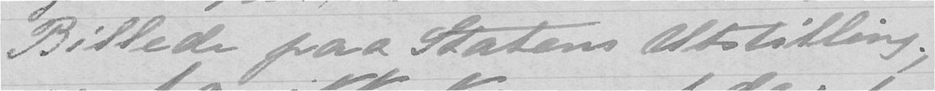Billede paa Statens Utstilling,
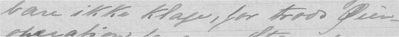bare ikke klage, for trods Øien-
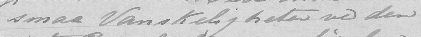smaa Vanskeligheter ved den
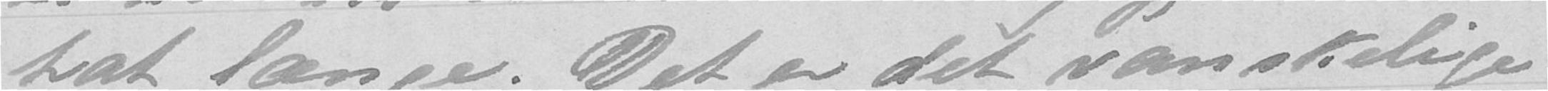hat længe. Det er det vanskelige
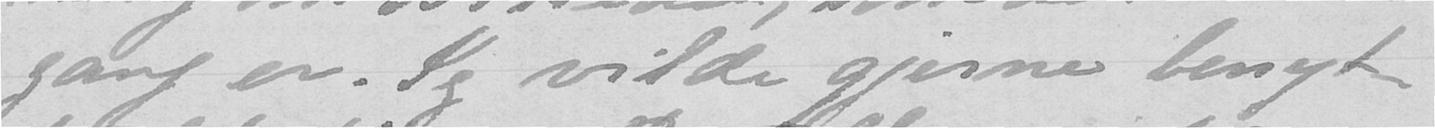gang er. Jeg vilde gjerne benyt-
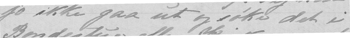jo ikke gaa ut og søke det i
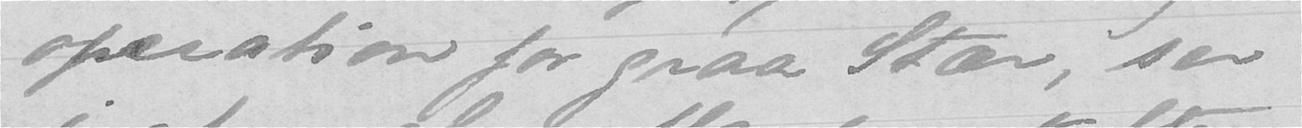operation for graa Stær, ser
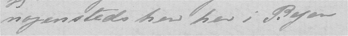nogenstede hen her i Byen
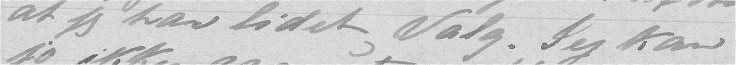at jeg har lidet Valg. Jeg kan
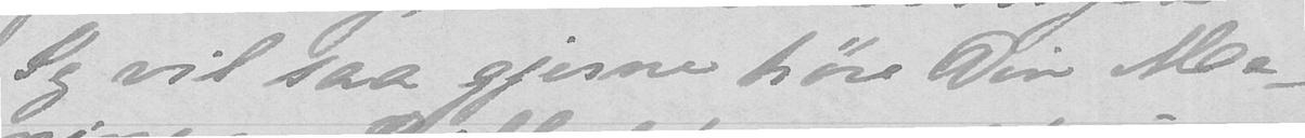Jeg vil saa gjerne høre Din Me-
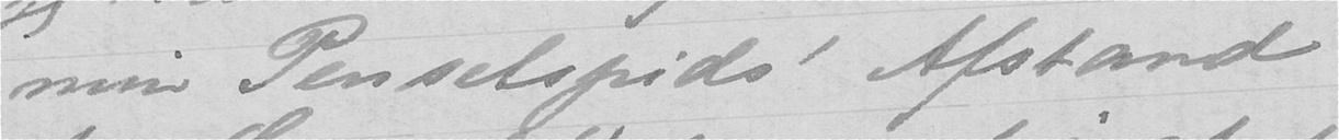min Penselspids' Afstand
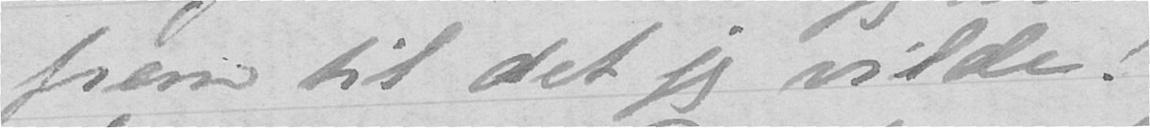frem til det jeg vilde!
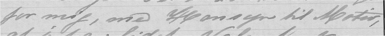for mig, med Hensyn til Motiv,
